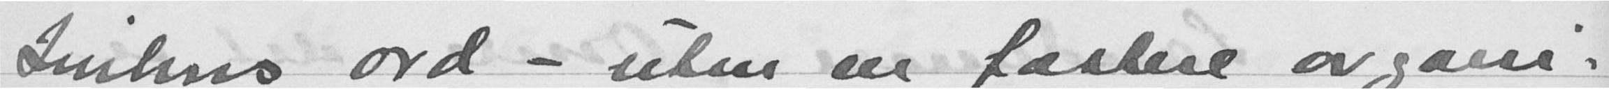Smlins ord - uten en fastere organi-
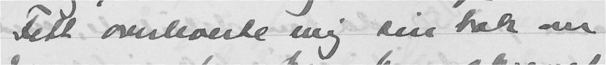Ditt overleverte mig sin bok om
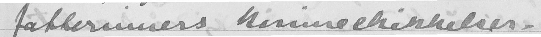atterinners kvinneskikkelser.
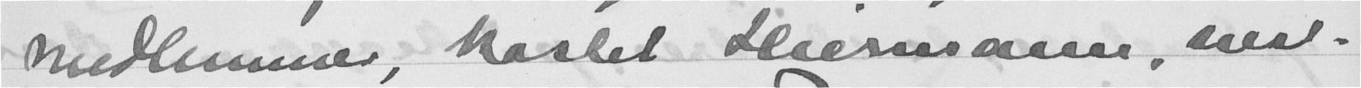medlemmer, kastet Hiermann, nest-
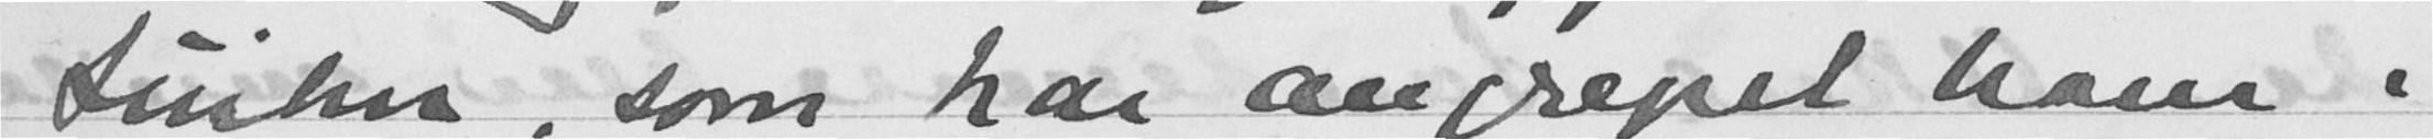Zusbiu, som har angrepet ham i
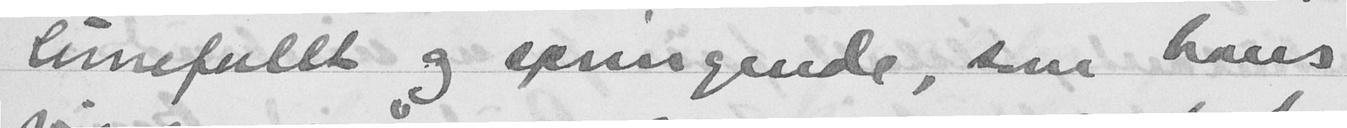lunefullt og springende, som hans
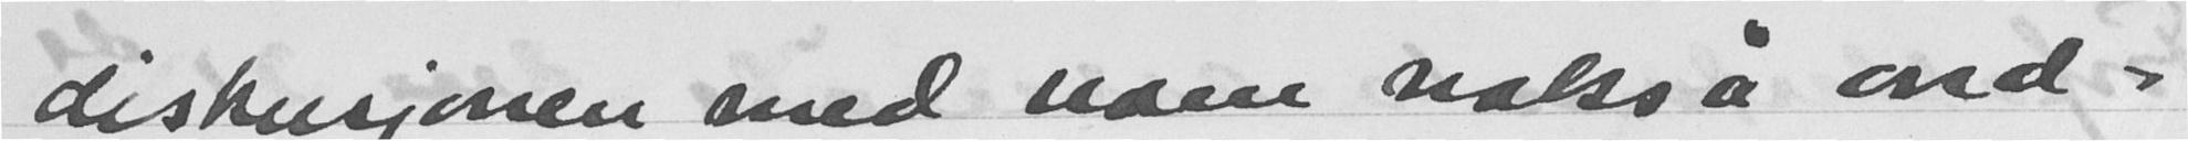diskusjonen med noen nokså ond-
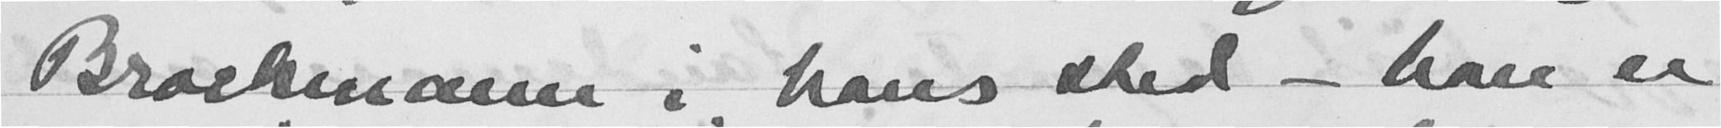Brockmann i hans sted - han er
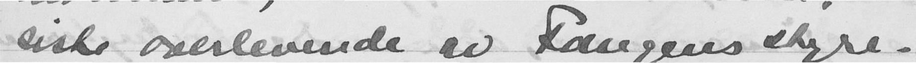siste overlevende av Fangens styre.
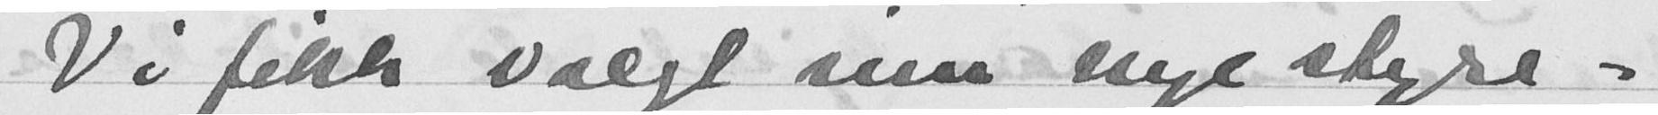Vi fikk valgt inn nye styre-
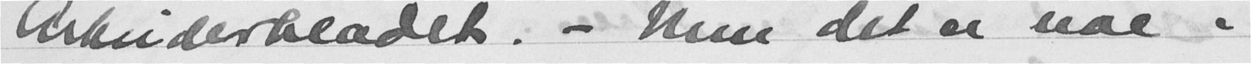Arbeiderbladet. - Men det er noe i
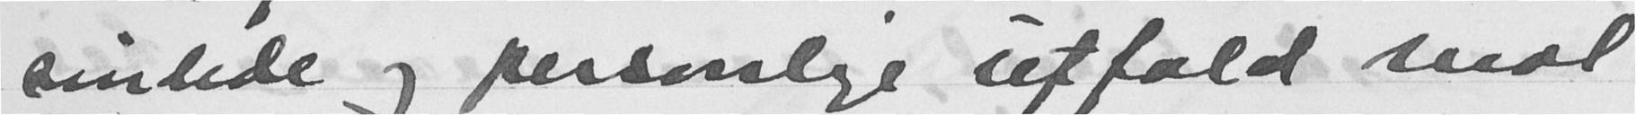sindede og personlige utfald mot
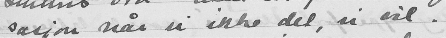sasjon når vi ikke det, vi vil.
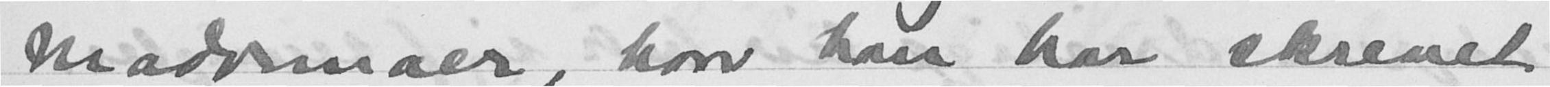madvimaer, hvor han har skrevet
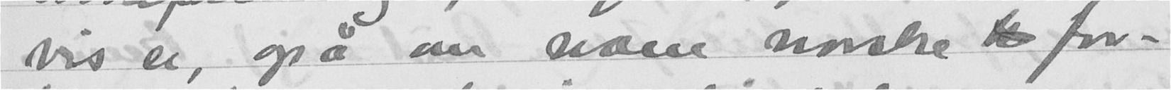vis er, også om nære norske  for-
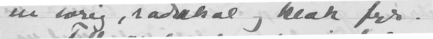en ivrig, radikal og klok fyr.
